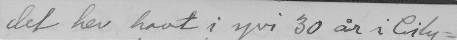det hev havt i yvi 30 år i City-
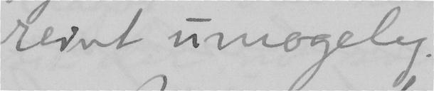reint umogelig.
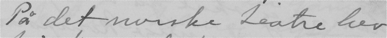På det norske teatre hev
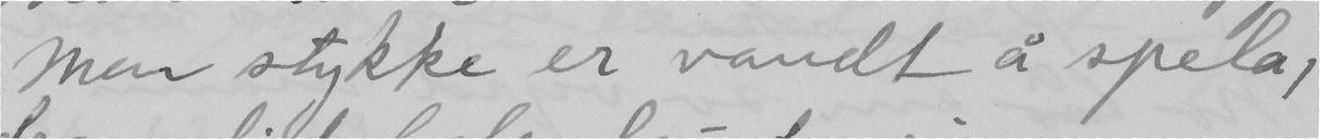Men stykke er vandt å spela,
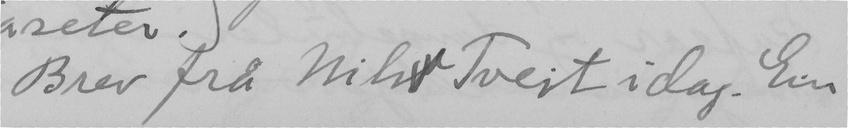Brev frå Nils Tveit idag. Ein
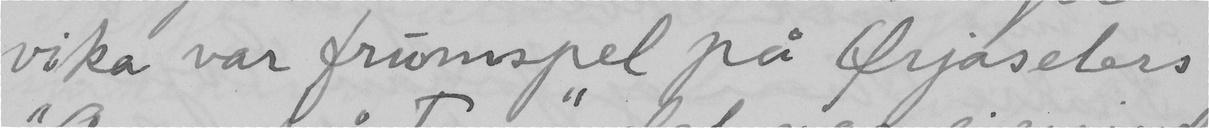vika var frumspel på Ørjaseters
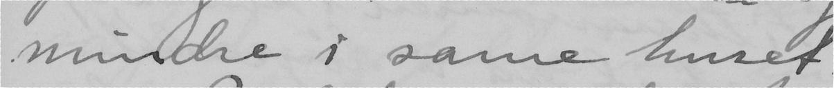mindre i same huset.
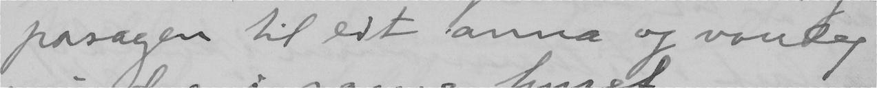pasagen til eit anna og vonleg
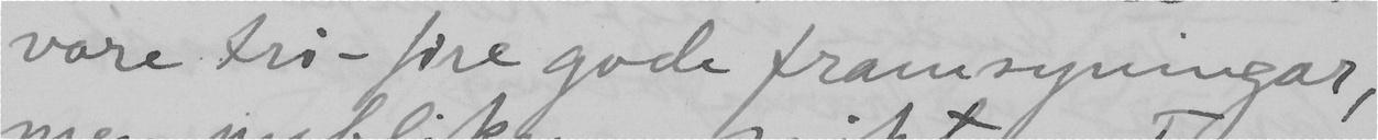vore tri-fire gode framsyningar,
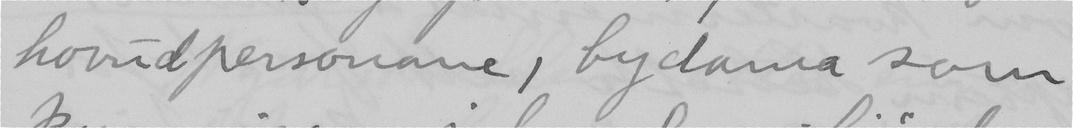hovudpersonane, bydama som
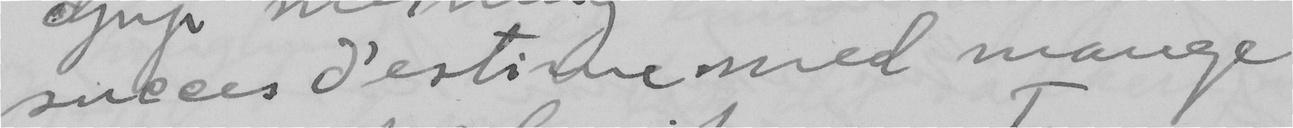success d'estime med mange
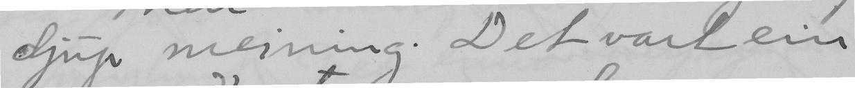djup meining. Det vart ein
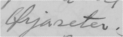Ørjaseter.
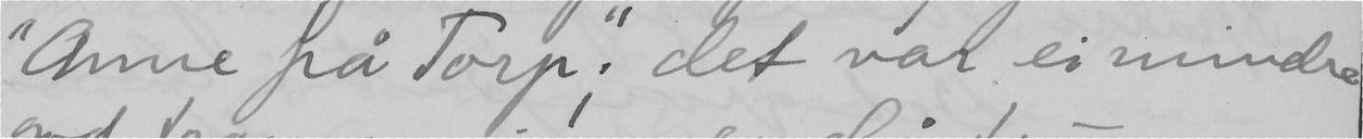"Anne på Torp"; det var ei mindre
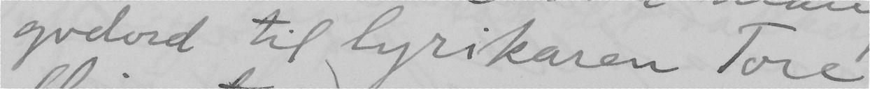godord til lyrikaren Tore
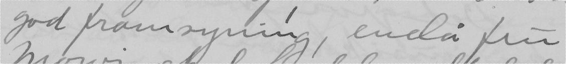god framsyning, endå fru
